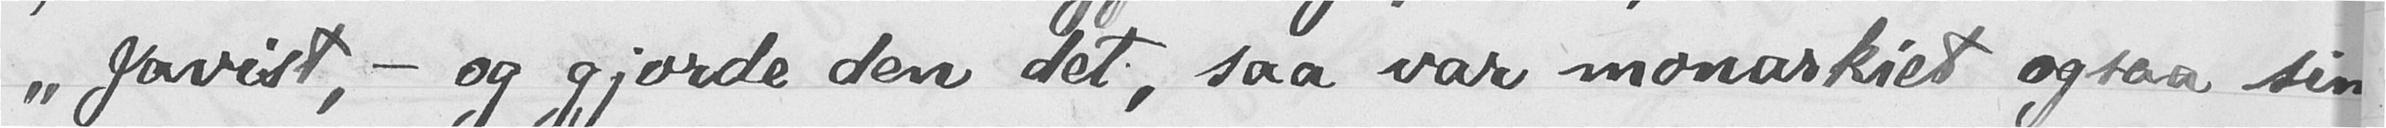"Javist, - og giorde den det, saa var monarkiet ogsaa sim-
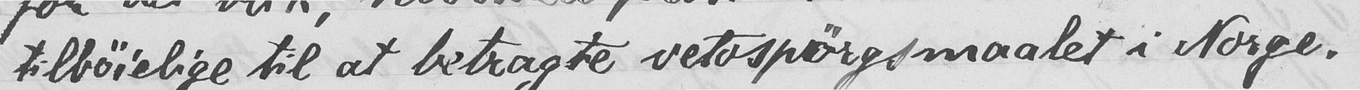tilbøielige til at betragte vetospørgsmaalet i Norge.
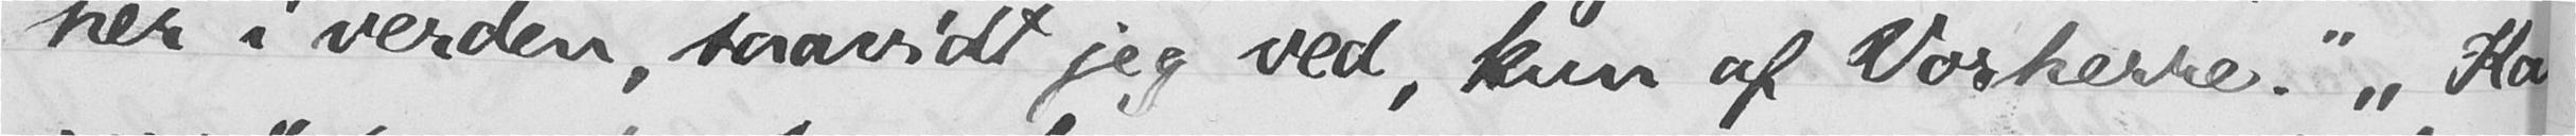her i verden, saavidt jeg ved, kun af Vorherre." "Kan
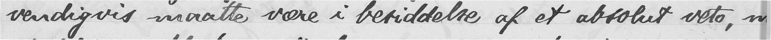vendigvis maatte være i besiddelse af et absolut veto, men
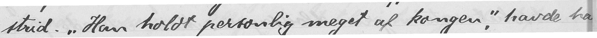strid. "Han holdt personlig meget af kongen", havde han
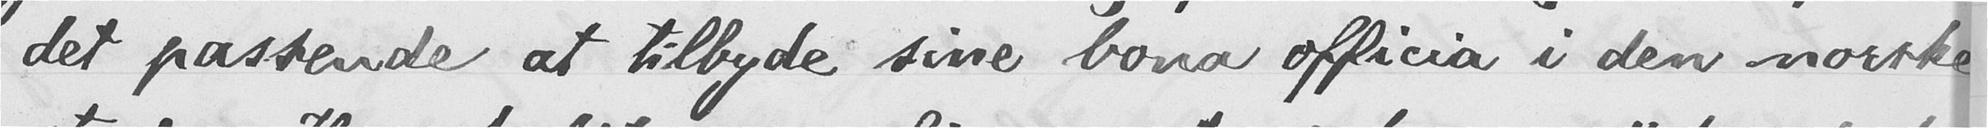det passende at tilbyde sine bona officia i den norske
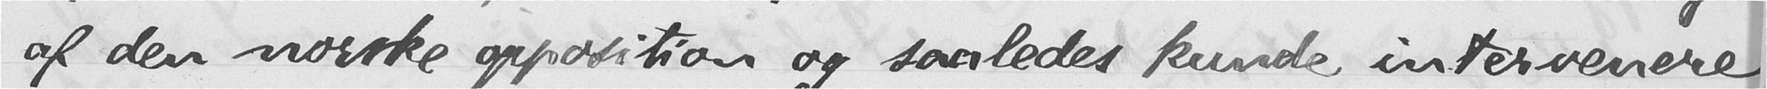af den norske opposition og saaledes kunde intervenere
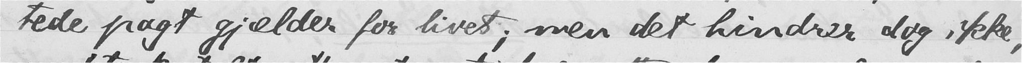tede pagt gjælder for livet; men det hindrer dog ikke, at
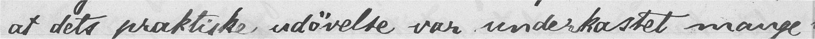at dets praktiske udøvelse var underkastet mange be-
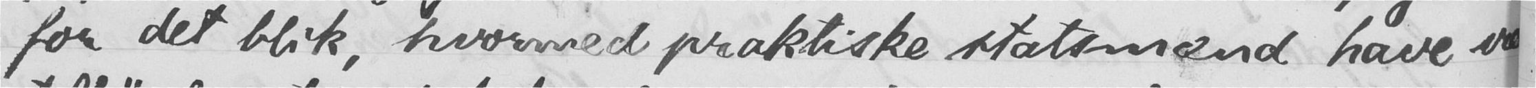for det blik, hvormed praktiske statsmænd have været
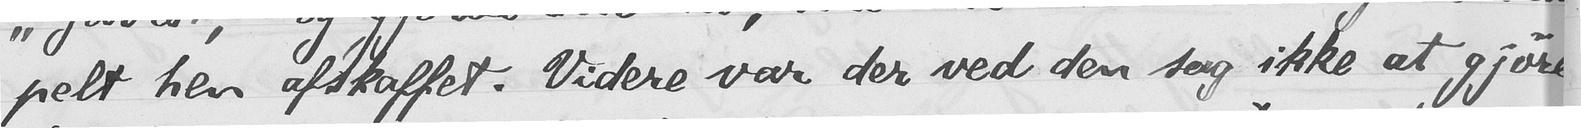pelt hen afskaffet. Videre var der ved den sag ikke at gjøre."
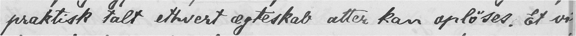praktisk talt ethvert ægteskab atter kan opløses. Et virke-
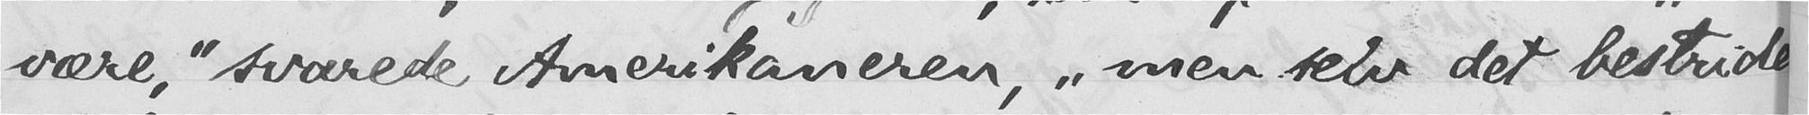være," svarede Amerikaneren, "men selv det bestrides
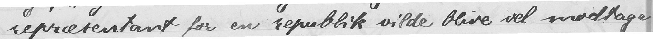repræsentant for en republik vilde blive vel modtaget
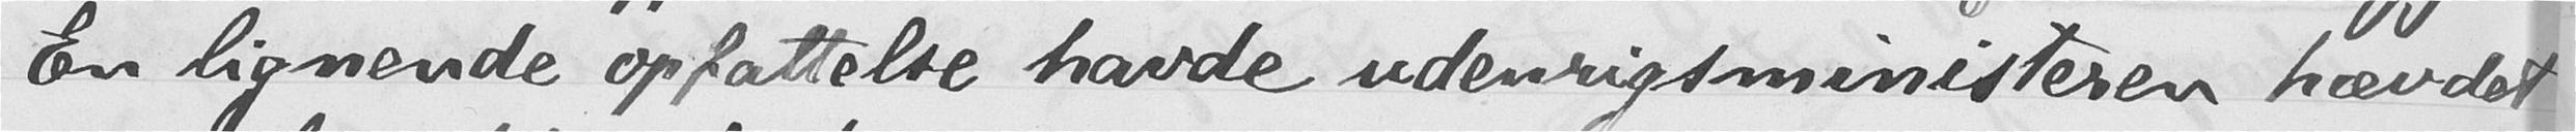En lignende opfattelse havde udenrigsministeren hævdet
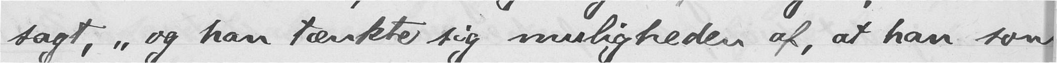sagt, "og han tænkte sig muligheden af, at han som
